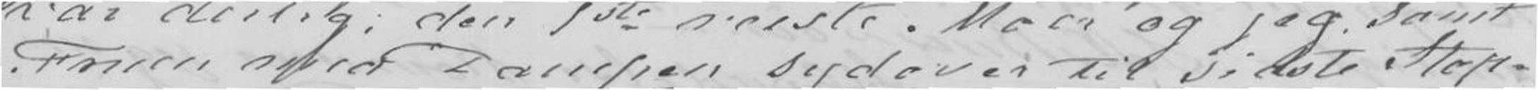Fruen med Dampen sydover til sidste Stop-
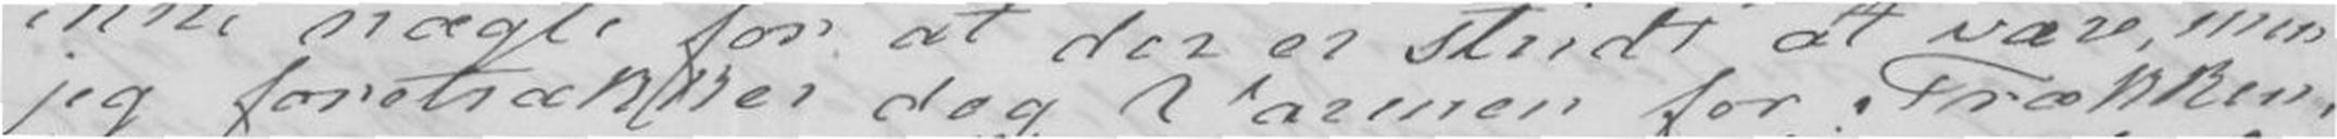jeg foretrækker dog Varmen for Trækken
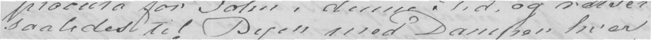saaledes til Byen med Dampen hver
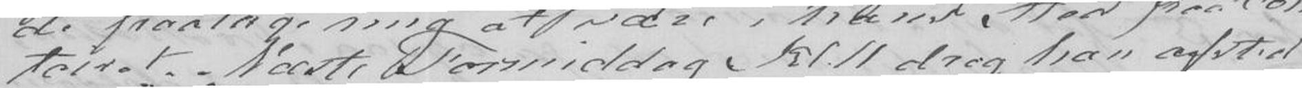toiret Næste Formiddag Kl.11 drog han afsted
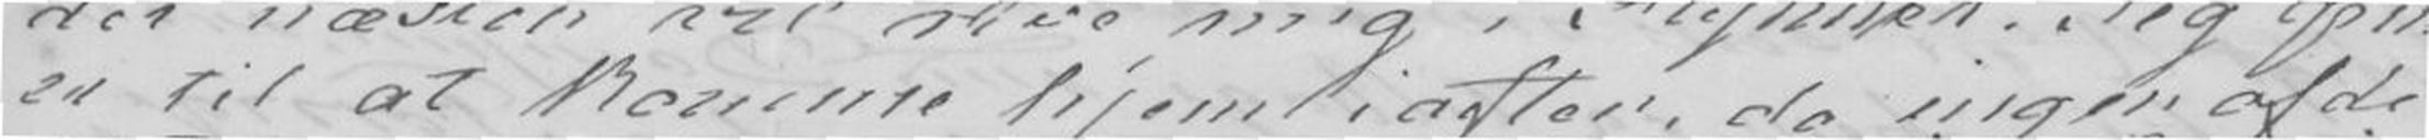er til at komme hjem i aften, da ingen af de
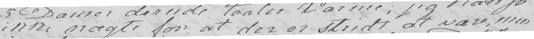ikke nægte for at der er stridt at være, men
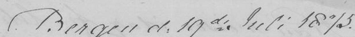Bergen d. 19de. Juli 1875.
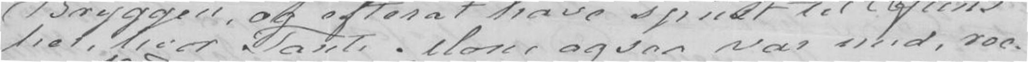her, hvor Tante Mone ogsaa var med, roe-
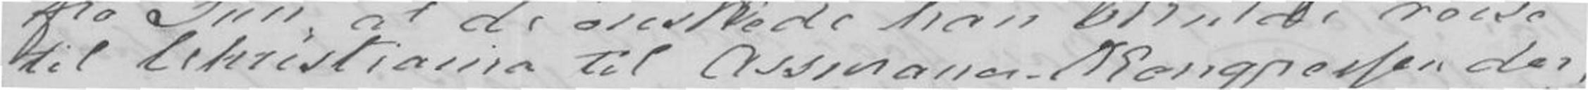til Christiania til Assurance-Kongressen der,
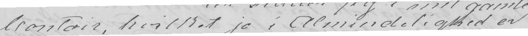Contoir, hvilket jo i Almindelighed er
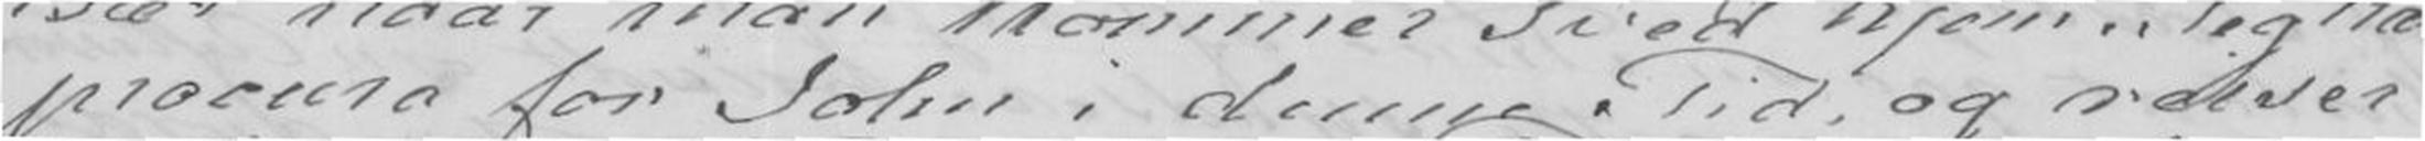procure for John i denne Tid, og reiser
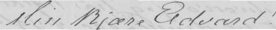Min kjære Edvard!
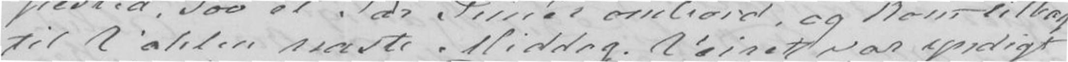til Vahlen næste Middag. Veiret var yndigt
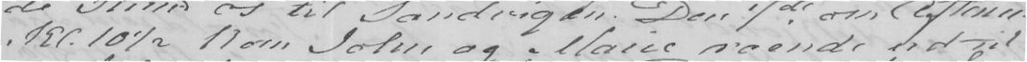Kl.10½ kom John og Marie roende ud til
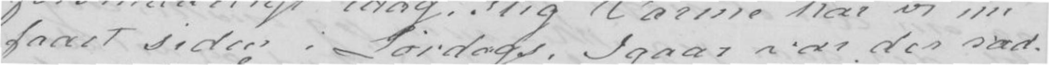faaet siden i Lørdags. Igaar var der sæd-
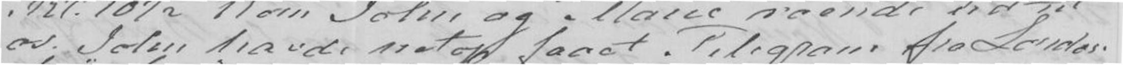os. John havde netop faaet Telegram fra London
